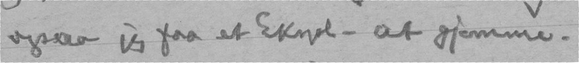ogsaa jeg faa et Ekspl. at gjemme.
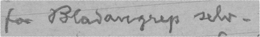for Bladangrep selv.
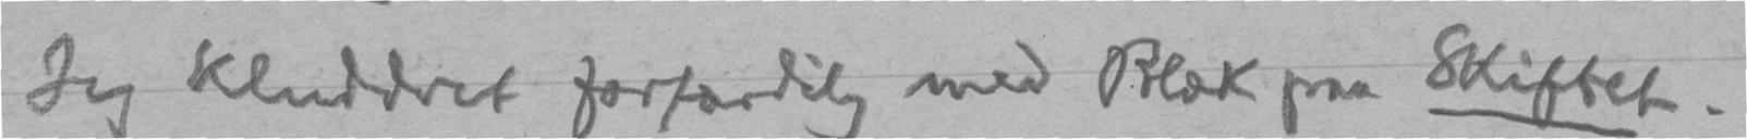Jeg kluddret forfærdilg med Blæk paa Skiftet.
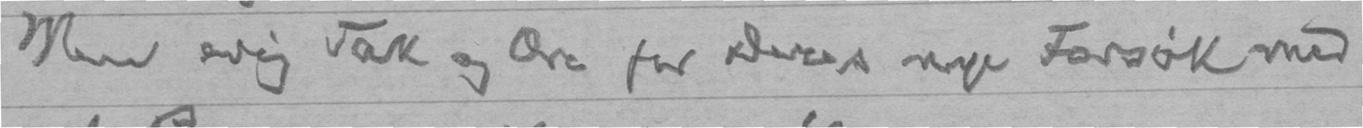Men evig Tak og Ære for Deres nye Forsøk med
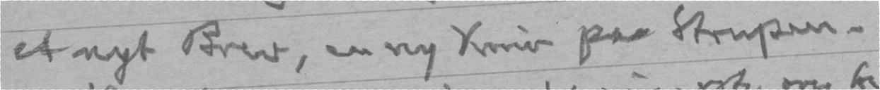et nyt Brev, en ny Kniv paa Strupen.
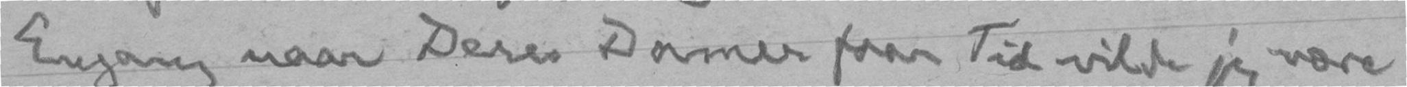Engang naar Deres Damer faar Tid vilde jeg være
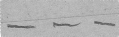- - -
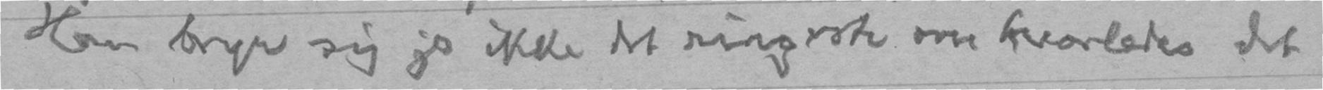Han bryr sig jo ikke det ringeste om hvorledes det
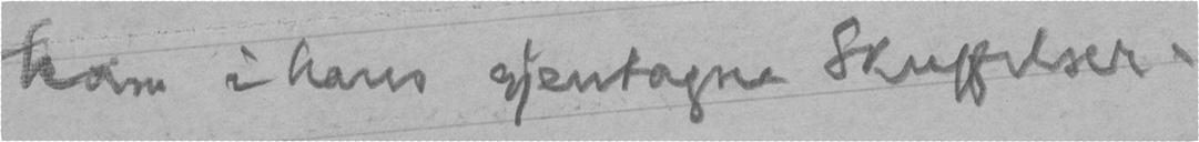ham i hans gjentagne Skuffelser.
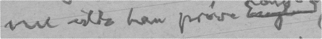nu vilde han prøve England
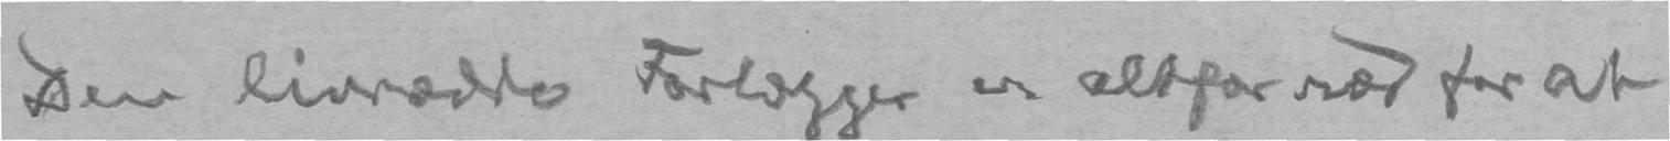Den livrædde Forlægger er altfor ræd for at
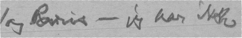og Paris - jeg har ikke
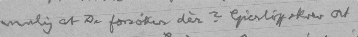mulig at De forsøker dèr? Gierløff skrev at
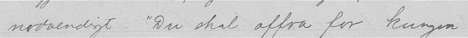nødvendigt. "Du skal offra for kungen
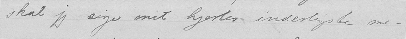skal jeg sige mit hjertes inderligste me-
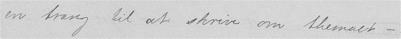en trang til at skrive om themaet -
